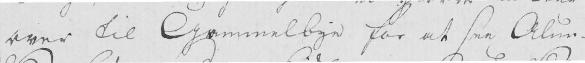over til Gammelbye for at see Alun-
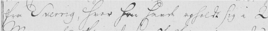fra Sverrig, hvor han havde opholdt sig i 2
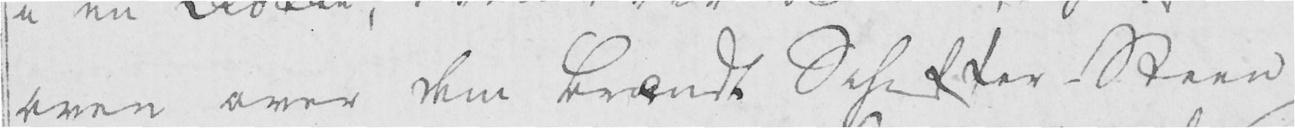oven over dem brændt Schiffer-Steen
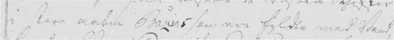i store aabne Bazins som ere fyldte med Vand,
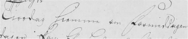Tirsdag hiemme om Formiddagen
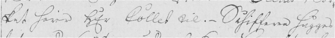ket hører Hr Collet til. - Schifferne hugges
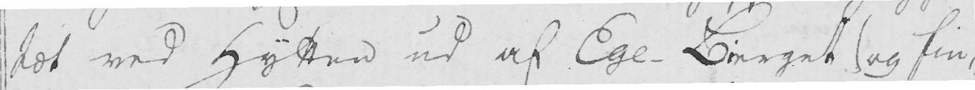tæt ved Hytten ud af Ege-Bierget og fin-
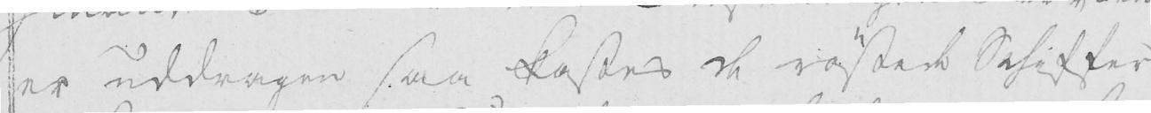er uddragen saa kastes de røstede Schiffer
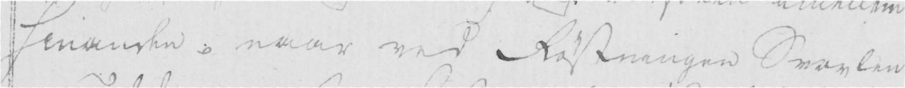hinanden; naar ved Røstningen Svovlen
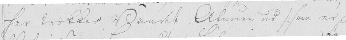her trækker Vandet Alunen ud /: som er i
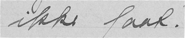ikke faat.
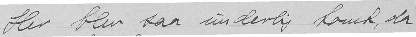Her blev saa underlig tomt, da
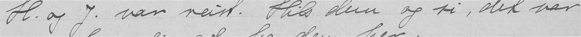H. og J var reist. Hils dem og si, det var
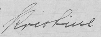Kristine
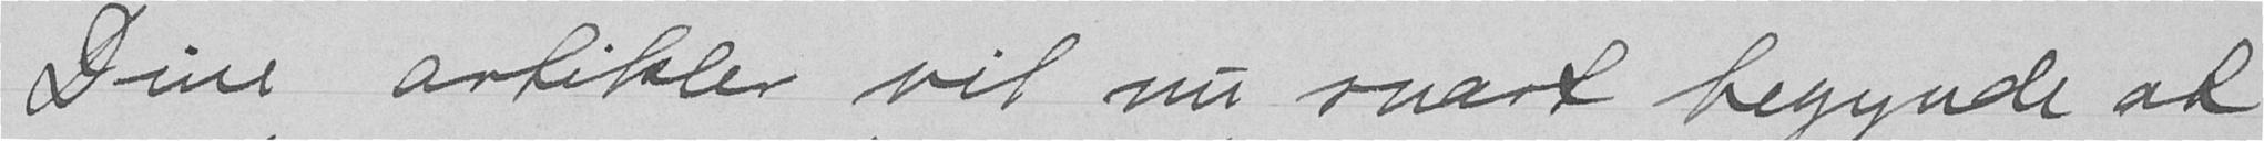Dine artikler vil nu snart begynde at
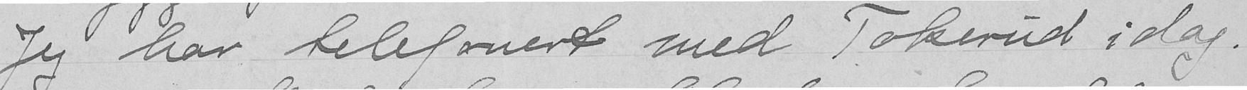Jeg har telefonert med Tokerud idag.
